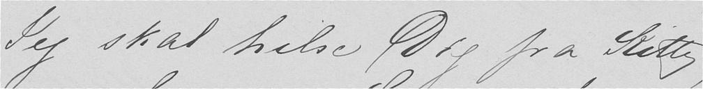Jeg skal hilse Dig fra Kitty,
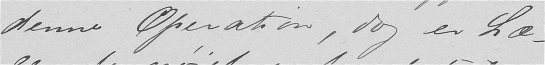denne Operation, dog er Læ-
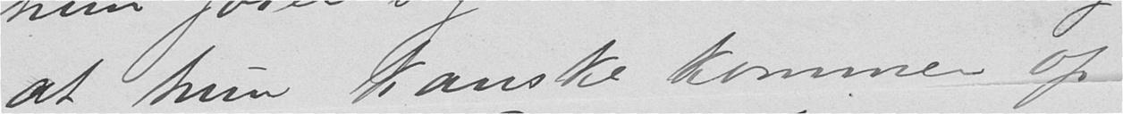at hun kanske kommer op
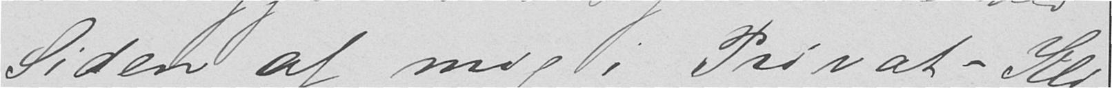Siden af mig i Privat-Kli-
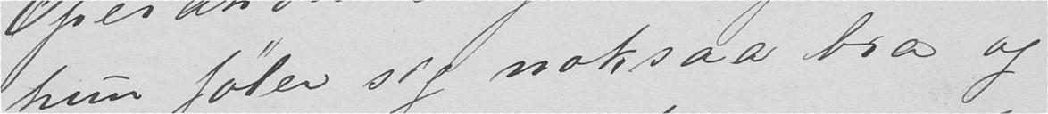hun føler sig noksaa bra og
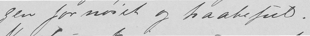gen fornøiet og haabefuld.
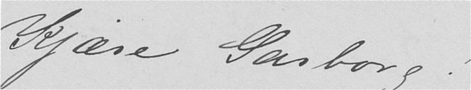Kjære Garborg!
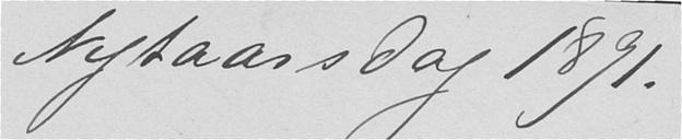Nytaarsdag 1891
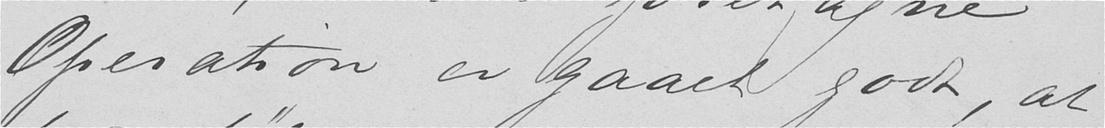Operation er gaaet godt, at
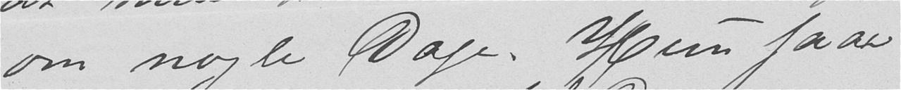om nogle Dage. Hun faar
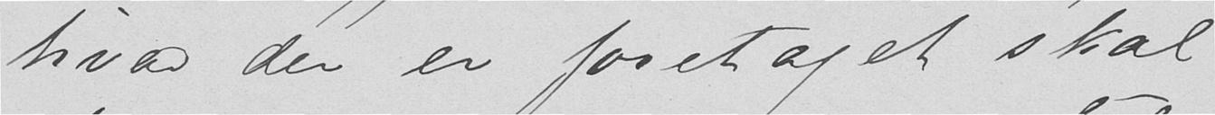hvad der er foretaget skal
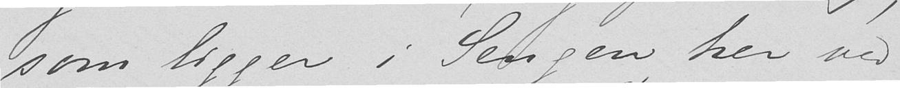som ligger i Sengen her ved
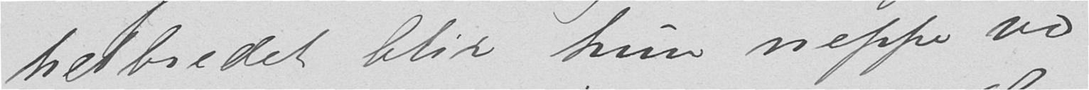helbredet blir hun neppe ved
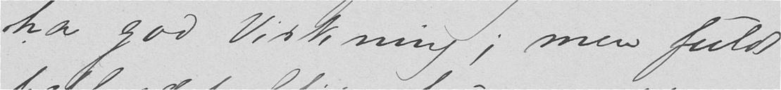ha god Virkning; men fuldt
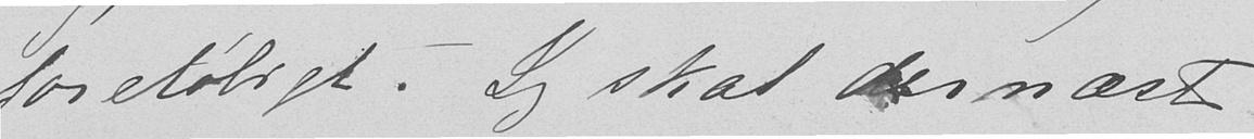foreløbigt. Jeg skal dernæst
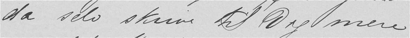da selv skrive til Dig mere
